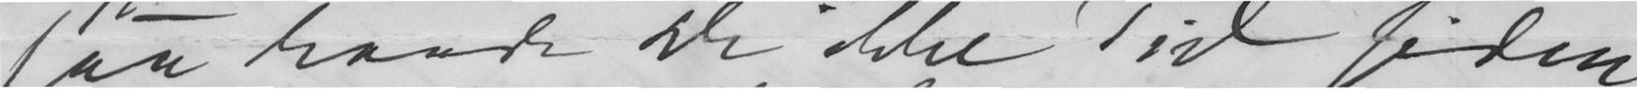saa havde De ikke Tid siden
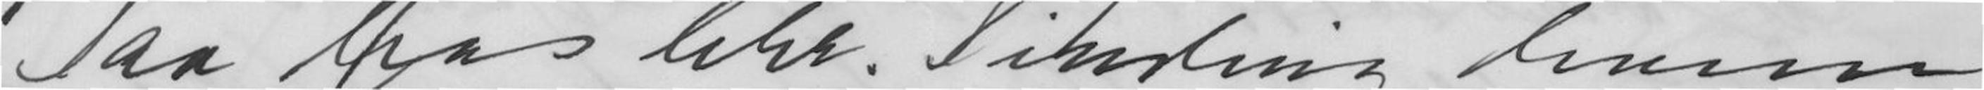Saa ha hrr. Sinding hvem
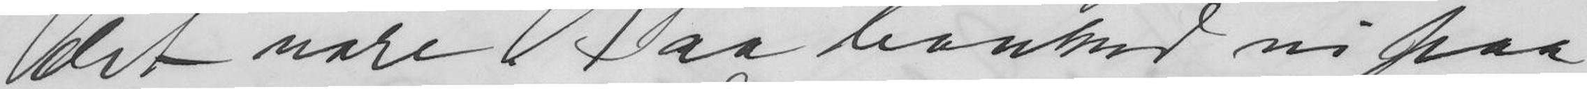det være. Saa banked vi paa
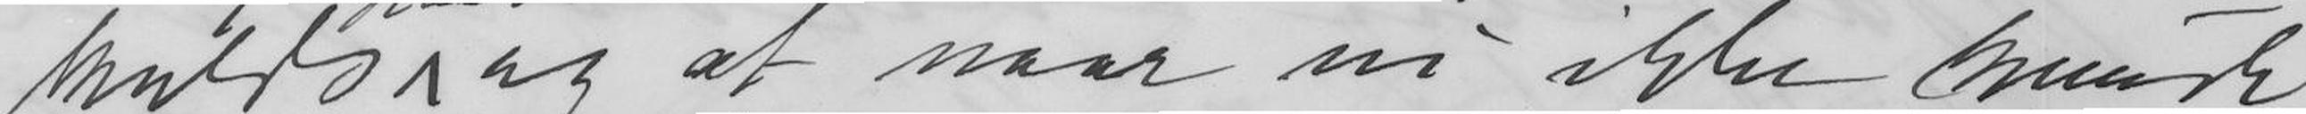kulds paa Dem og at naar vi ikke kunde
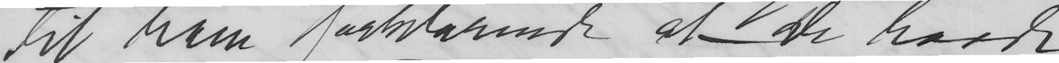til ham forklarede at De havde
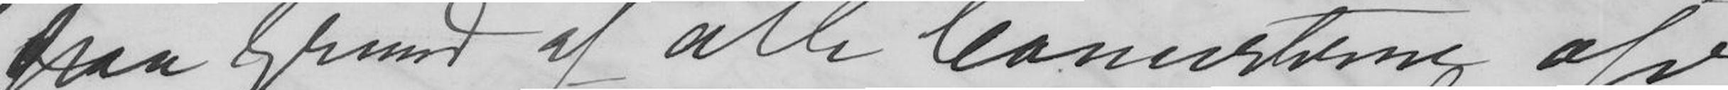paa Grund af alle Concerterne osv.
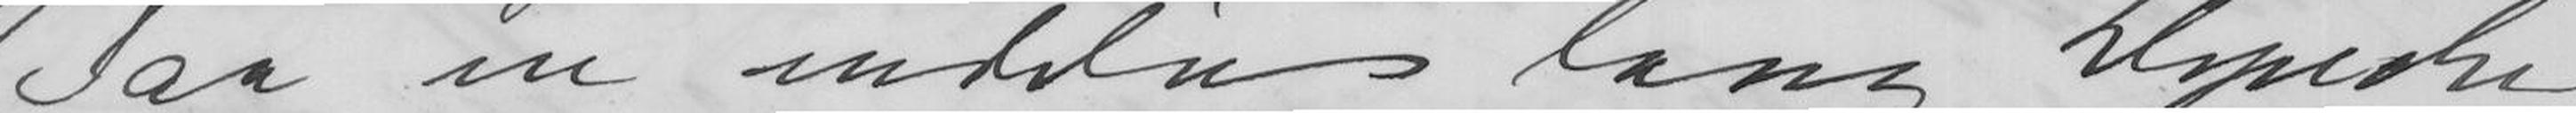Saa en endeløs lang Depeche
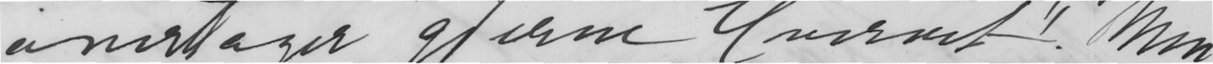overtager gjerne Hvervet. Men
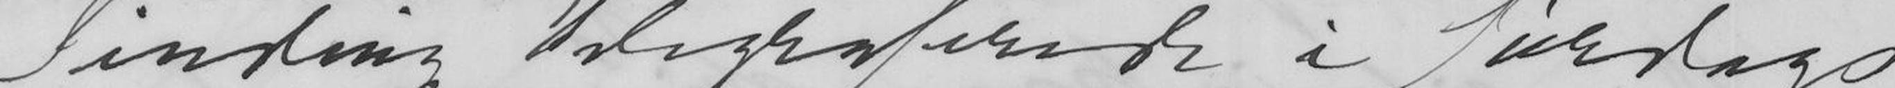Sinding telegraferede i Lørdags
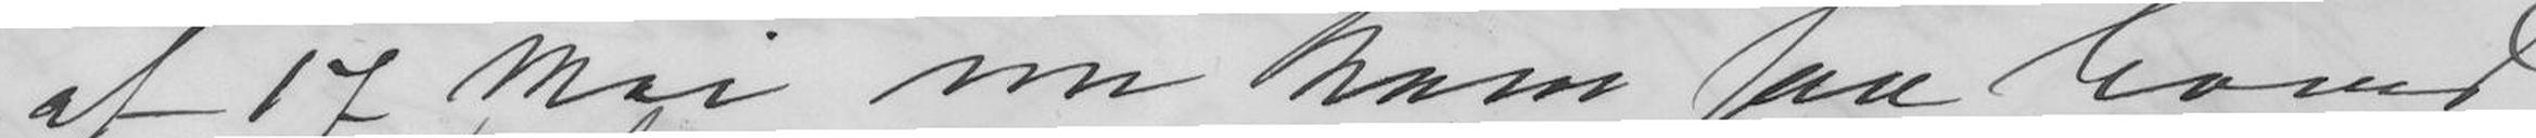at 17 Mai nu kom saa hoved-
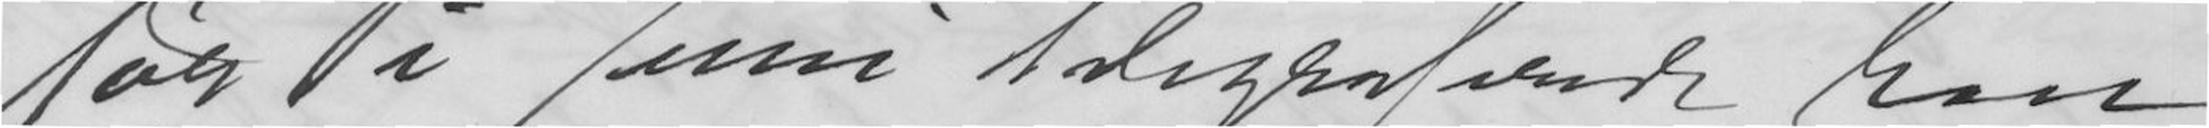før i Juni telegraferede han.
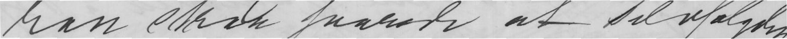han strax svarede at selvfølgelig
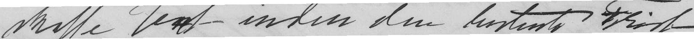skaffe Text inden den hostende Frist
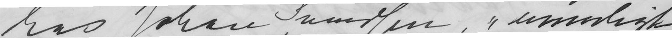hos Johan Svandsen, umuligt
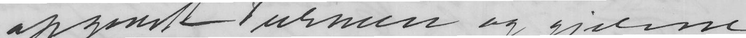opgivet Turneen og gjerne
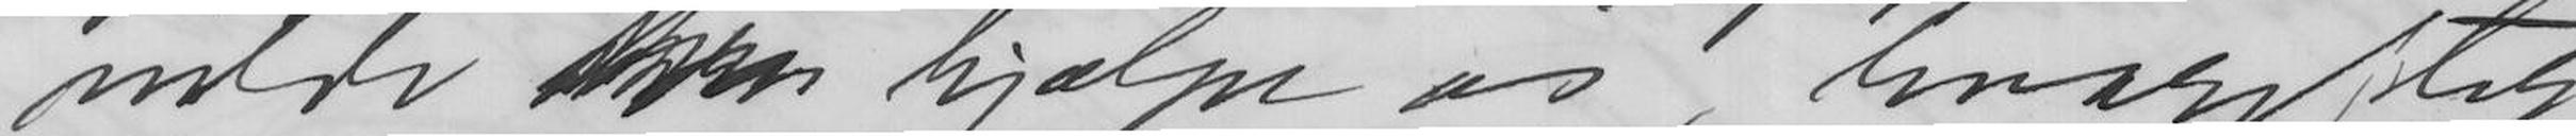vilde hjælpe os, hvorefter
